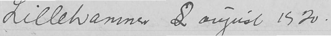Lillehammer 8. august 1920.
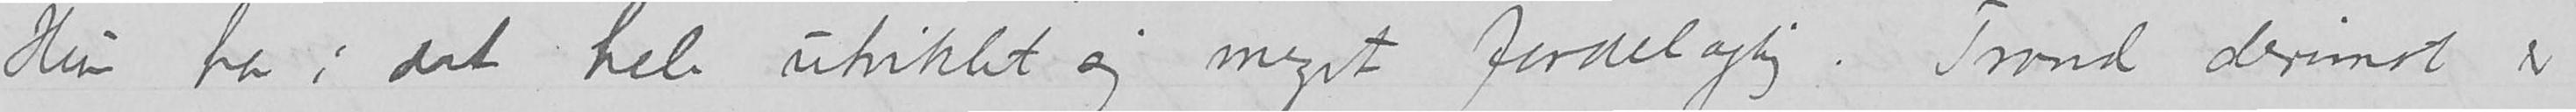Hun har i det hele utviklet sig meget fordelagtig.  Trond derimot er
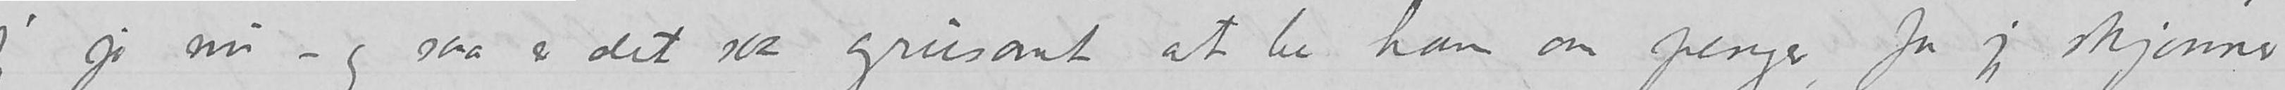jeg jo nu – og saa er det saa grusomt at be ham om penger, for jeg skjønner
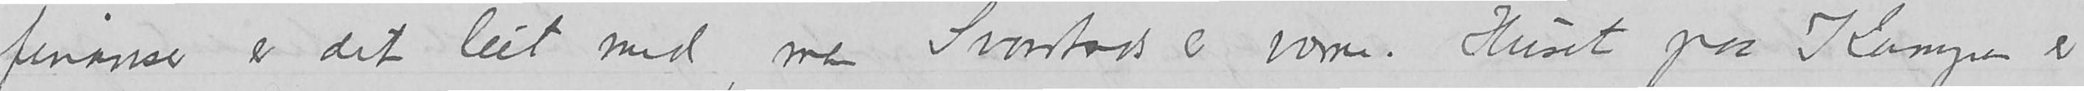finanser er det leit med, men Svarstads er verre.  Huset paa Kampen er
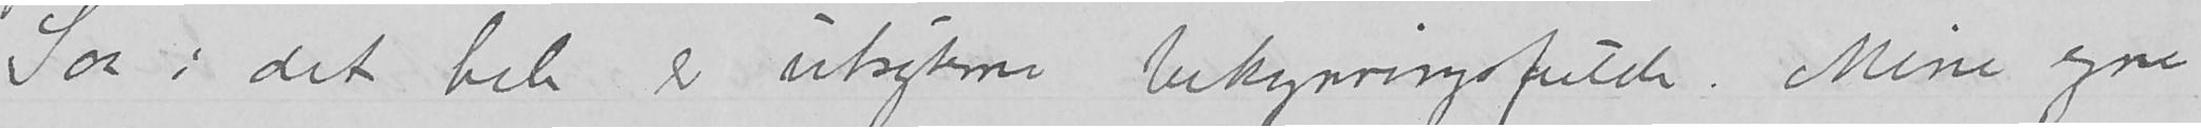Saa i det hele er utsigterne bekymringsfulde.  Mine egne
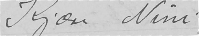Kjære Nini,
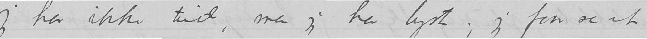jeg har ikke tid, men jeg har lyst;  jeg faar se at
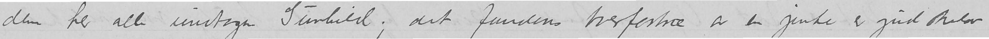dem her alle undtagen Gunhild; det fandens hespetræ el tverfortræ? av en jente er gudskelov
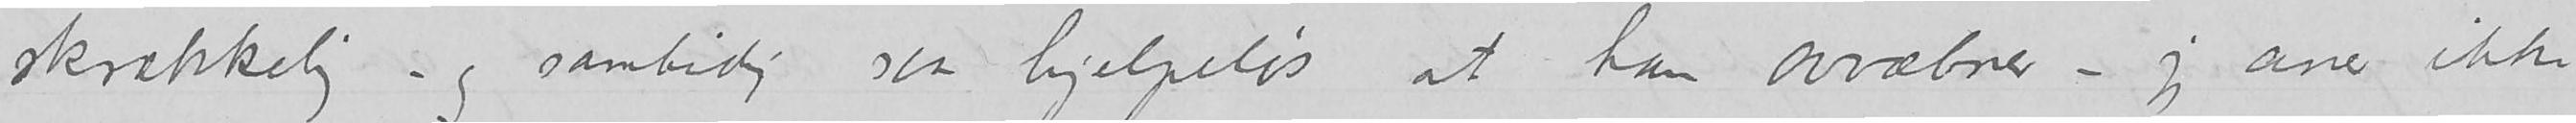skrækkelig – og samtidig saa hjelpeløs at han avvæbner – jeg aner ikke
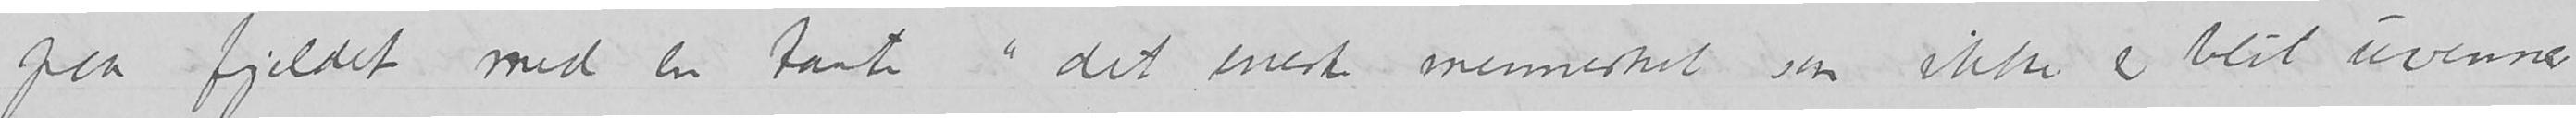paa fjeldet med en tante «det eneste mennesket som ikke er blit uvenner
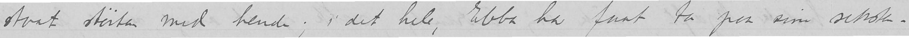staat stürten med hende; i det hele, Ebba har faat ta paa sine seksten-
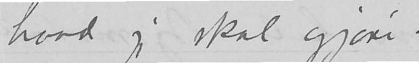hvad jeg skal gjøre.
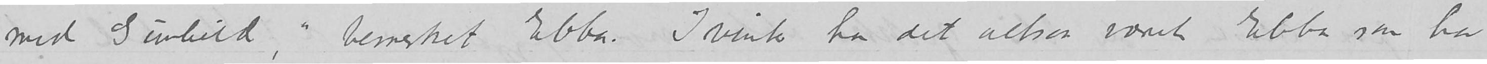med Gunhild,» bemerket Ebba.  I vinter har det altsaa været Ebba som har
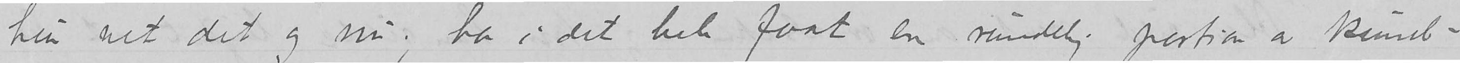hun vet det og nu;  har i det hele faat en rundelig portion av kund-
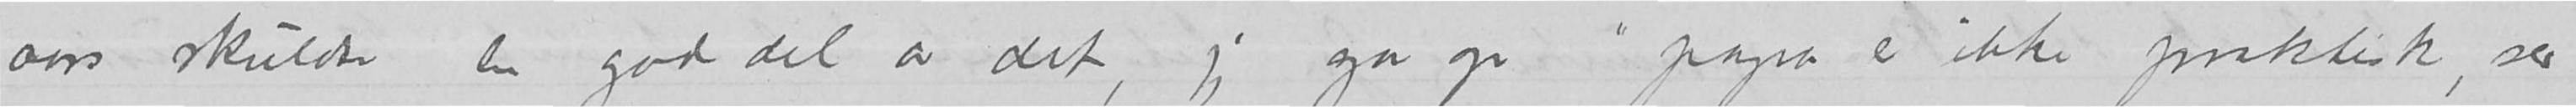aars skuldre en god del av det, jeg ga op «papa er ikke praktisk, ser
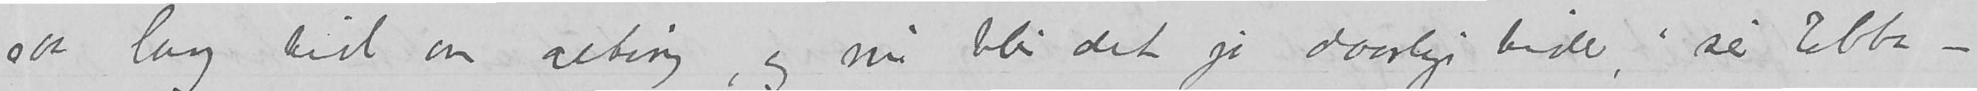saa lang tid om alting, og nu blir det jo daarlige tider,» sier Ebba –
